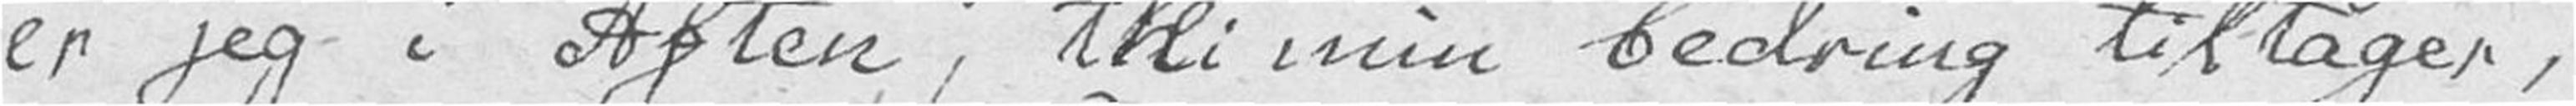er jeg i Aften, thi min bedring tiltager,
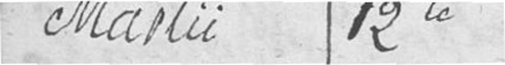Martii 12te
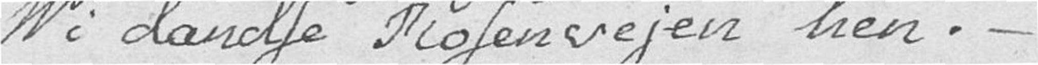Vi dandse Rosenvejen hen. –
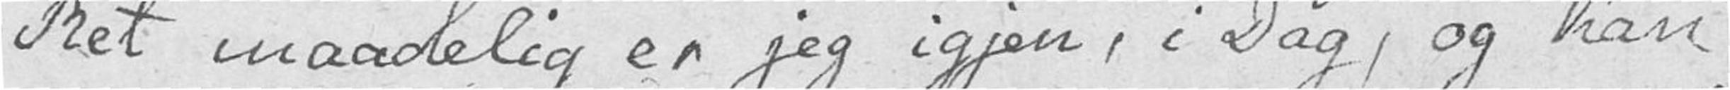Ret maadelig er jeg igjen, i Dag, og kan
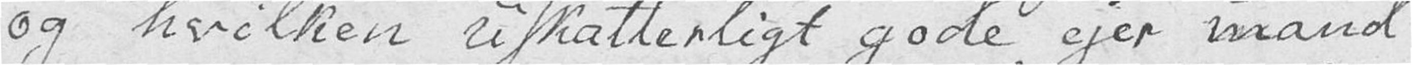og hvilken uskatterligt gode ejer mand
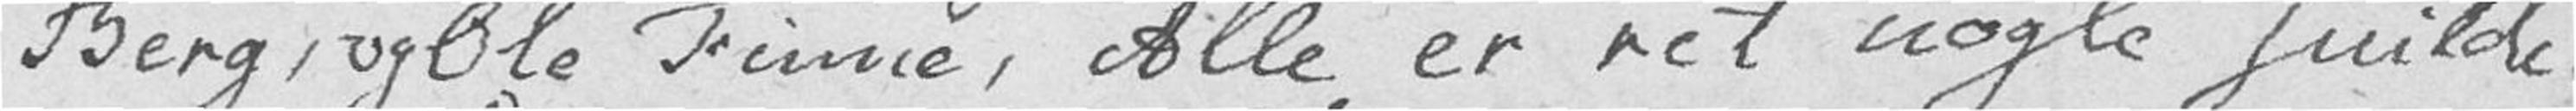Berg, og Ole Finne, Alle er ret nogle snilde
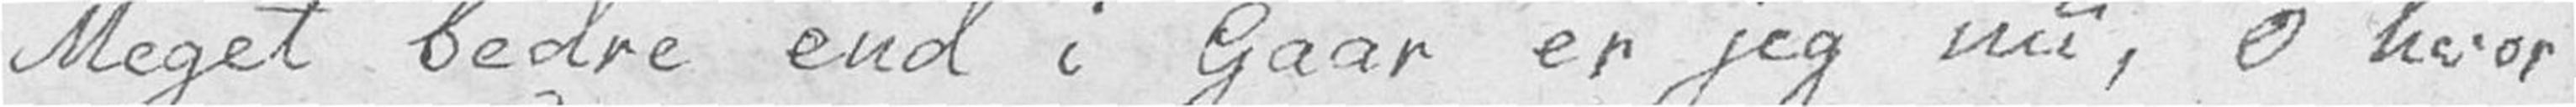Meget bedre end i Gaar er jeg nu, O hvor
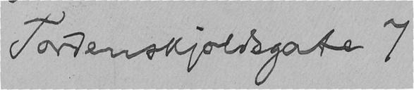Tordenskjoldsgate 7
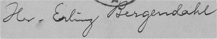Hr. Erling Bergendahl
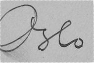Oslo
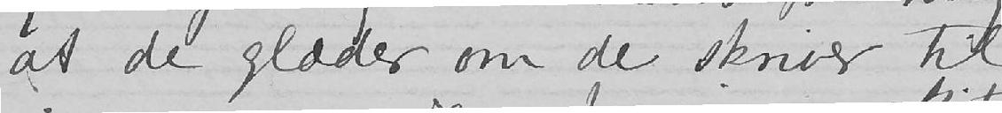at de glæder om de skriver til
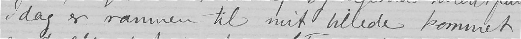Idag er rammen til mit billede kommet
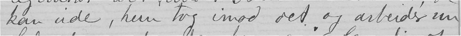kan vide, hun tog imod det og arbeider nu
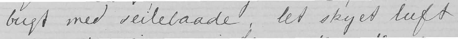bugt med seilebaade, let skyet luft
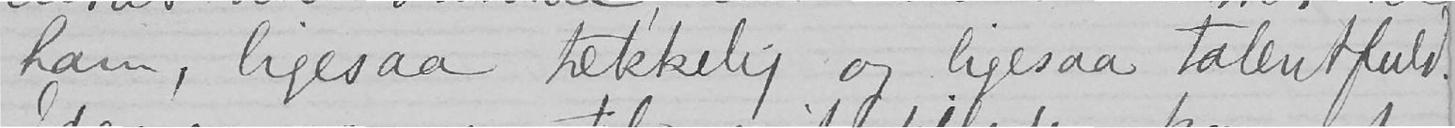ham, ligesaa tækkelig og ligesaa talentfuld.
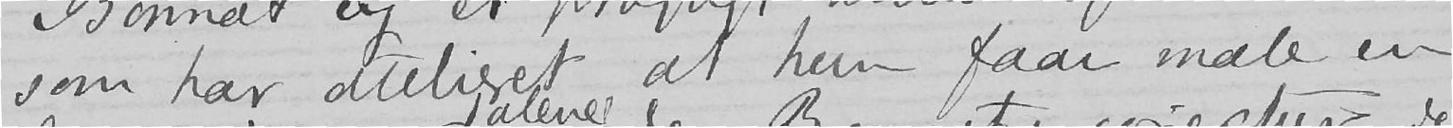som har atelieret at hun faar male en
